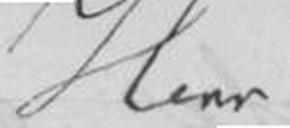Herr
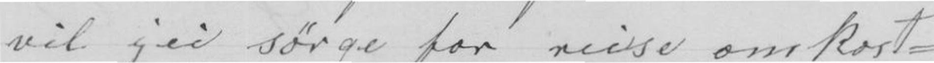vil jei sørge for reise omkost-
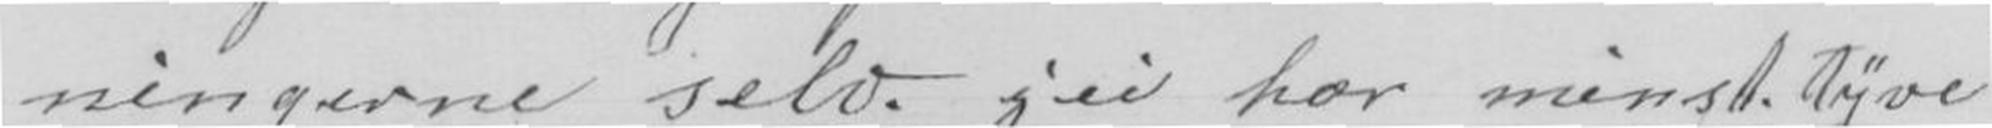ningerne selv. jei hor minst tyve
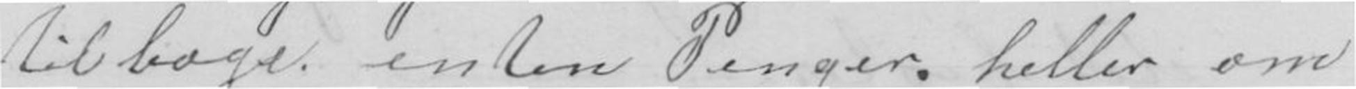tilbage enten Penger. heller om
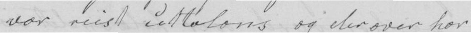vor reist uttalons og der over hor
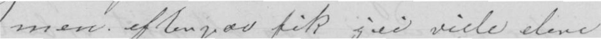men efterpaa fik jei vide dere
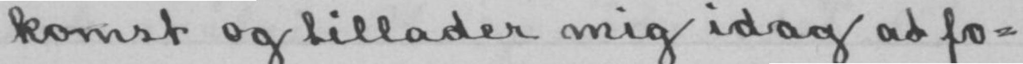komst og tillader mig idag at fo-
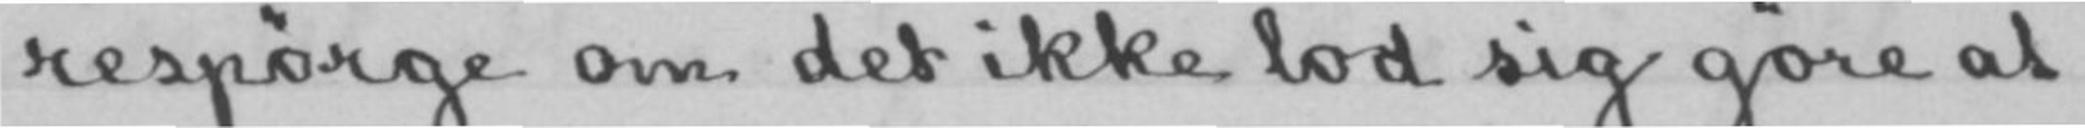respørge om det ikke lod sig gøre at
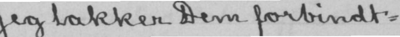Jeg takker Dem forbindt-
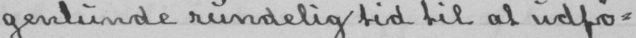genlunde rundelig tid til at udfø-
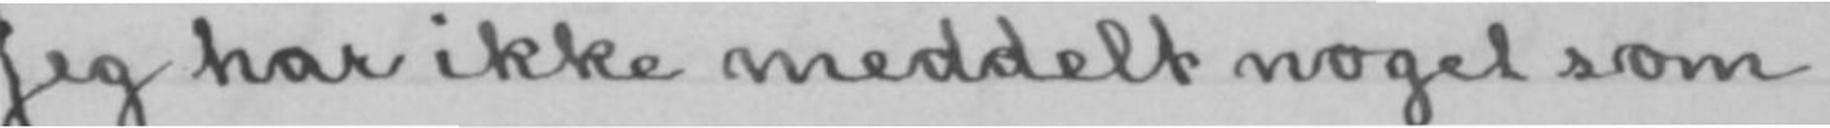Jeg har ikke meddelt noget som
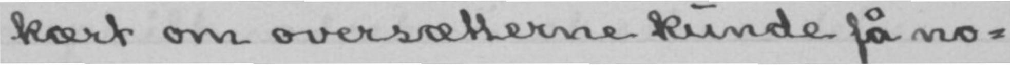kært om oversætterne kunde få no-
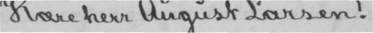Kære herr August Larsen!
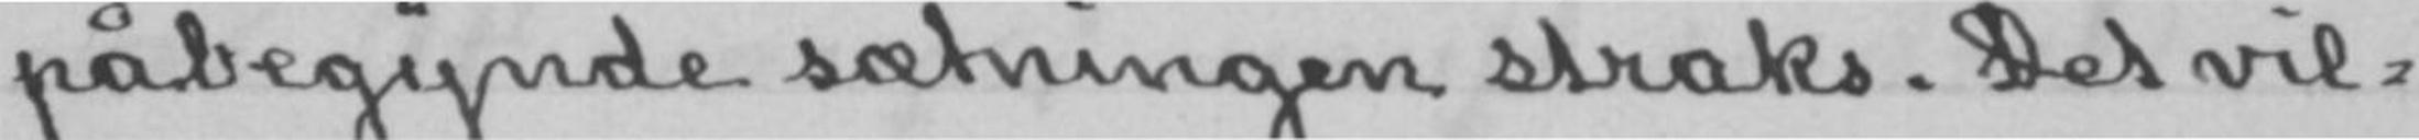påbegynde sætningen straks. Det vil-
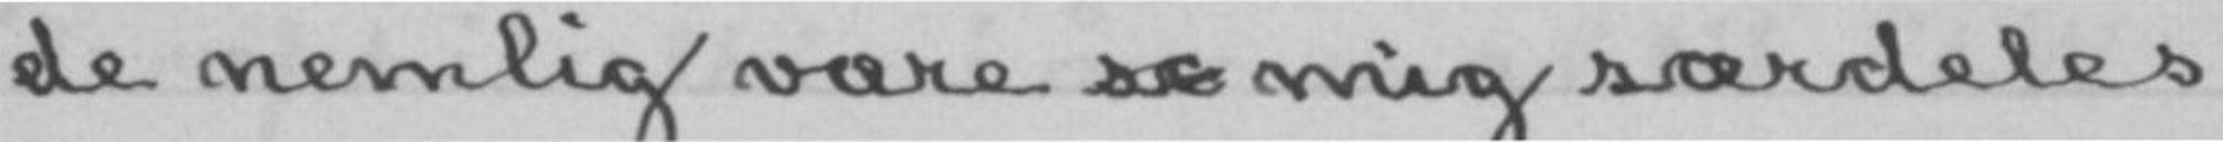de nemlig være s mig særdeles
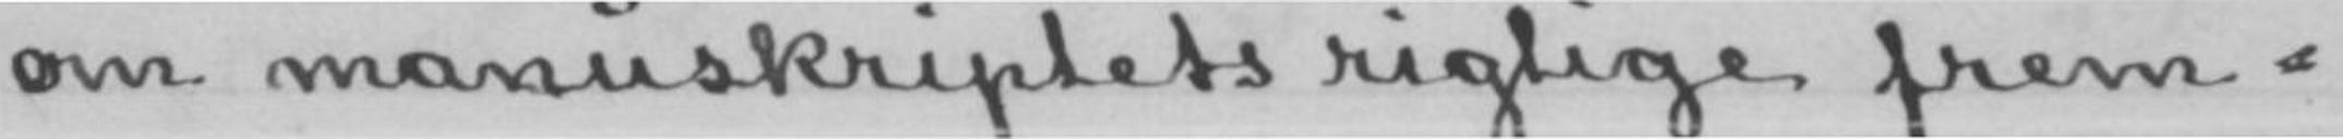om manuskriptets rigtige frem-
